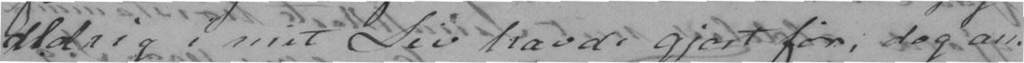aldrig i mit Liv havde gjort før, dog an-
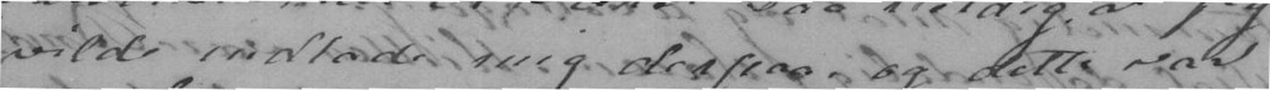vilde indlade mig derpaa, og dette var
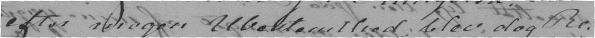efter megen Ubestemthed blev dog Re-
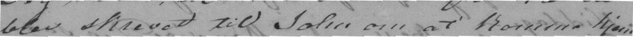blev skrevet til John om at komme hjem
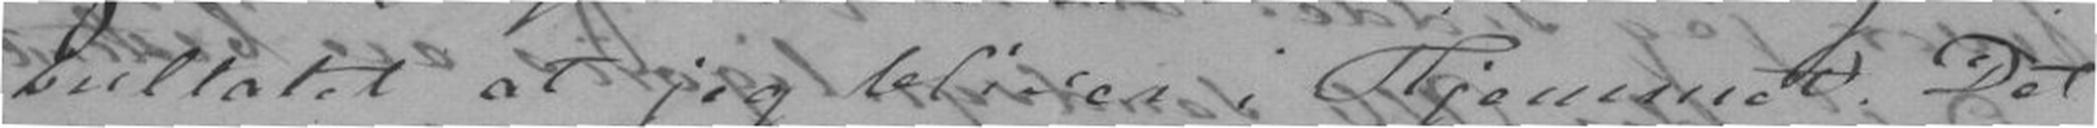sultatet at jeg bliver i Hjemmet. Det
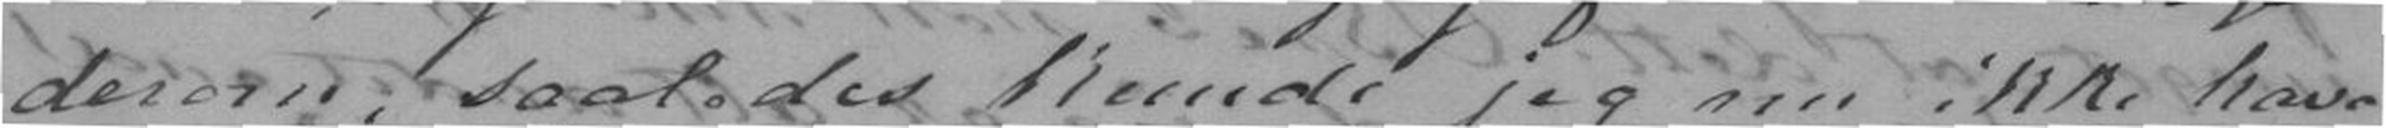derom, saaledes kunde jeg nu ikke have
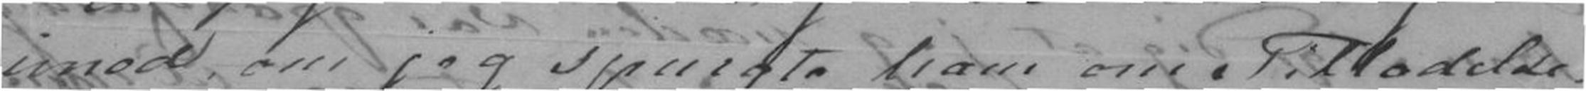imod, om jeg spurgte ham om Tilladelse.
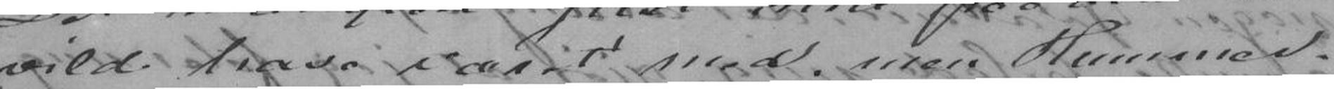vilde have været med, men Hummer-
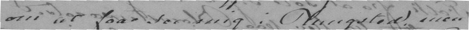om at faae see mig i Rungsted, men
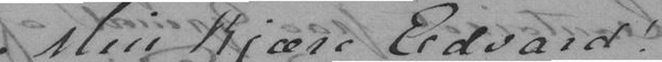Min kjære Edvard!
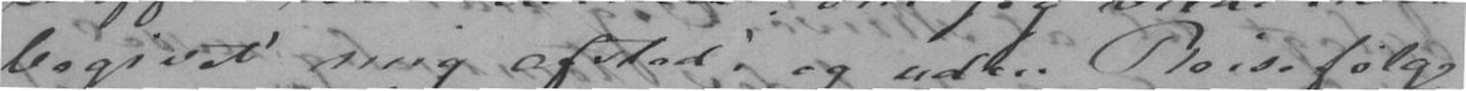begivet mig afsted, og uden Reisefølge
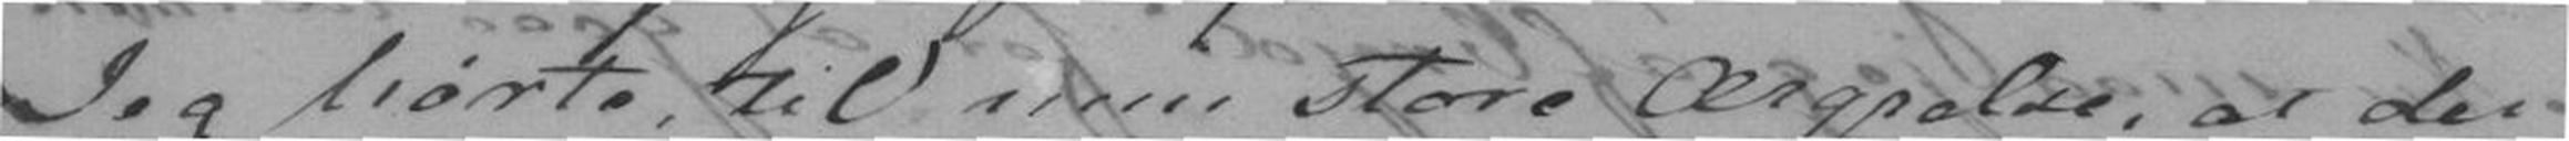Jeg hørte til min store Ærgrelse, at der
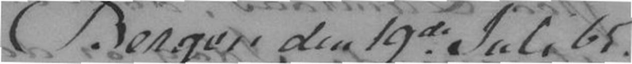Bergen den 19de Juli 65.
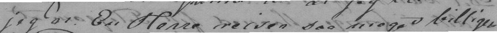jeg er. En Herre reiser saa meget billigere
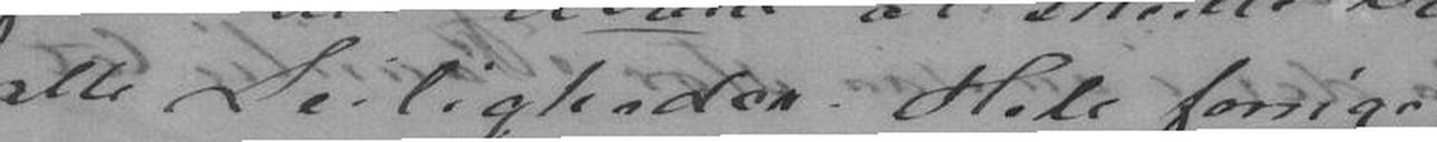alle Leiligheder. Hele forrige
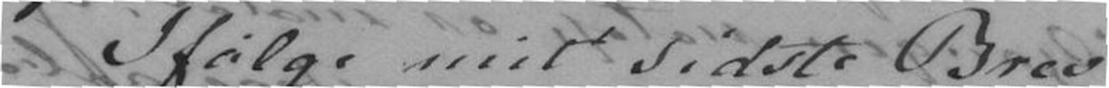Ifølge mit sidste Brev
